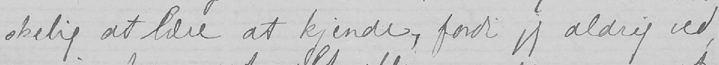skelig at lære at kjende, fordi jeg aldrig ved,
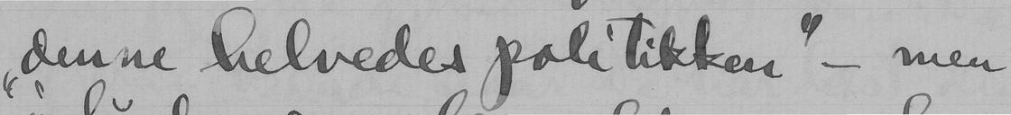"denne helvedes politikken" - men
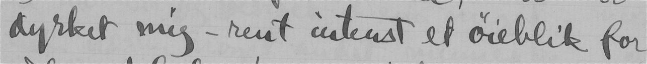dyrket mig - rent intenst et øieblik for
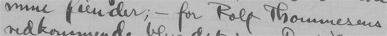mine fiender; - for Rolf Thommesens
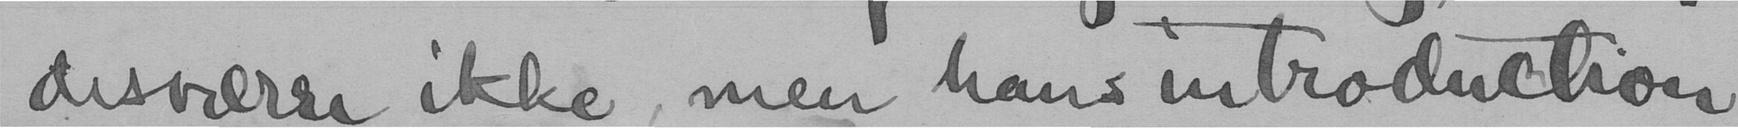desværre ikke, men hans introduction
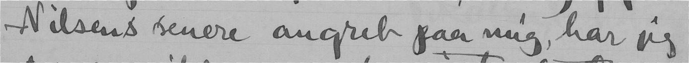Nilsens senere angreb paa mig, har jeg
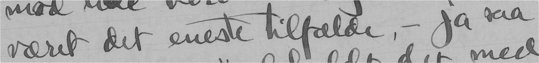været det eneste tilfælde - ja saa
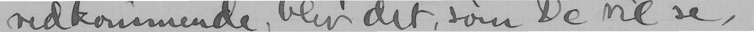vedkommende, blev det, som De vil se,
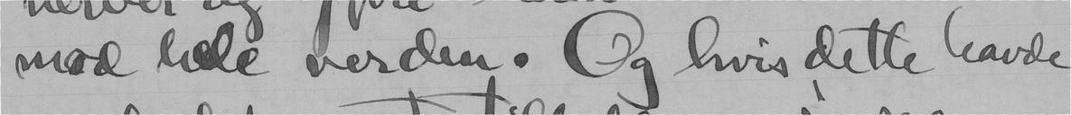mod hele verden. Og hvis dette havde
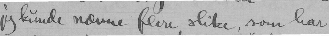jeg kunde nævne flere slike, som har
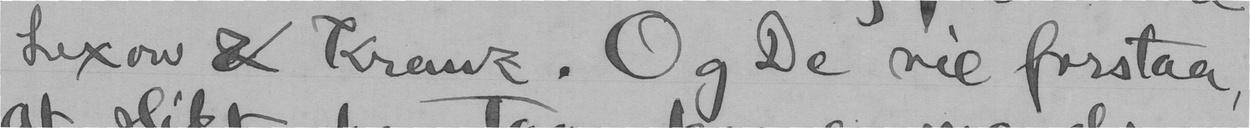Lexow & Kranz. Og De vil forstaa,
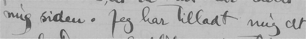mig siden. Jeg har tilladt mig at
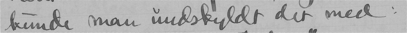kunde man undskyldt det med:
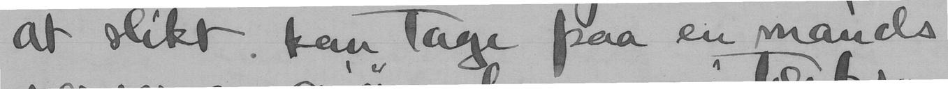at slikt kan tage paa en mands
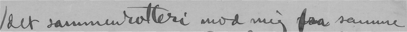det sammenrotteri mod mig fra samme
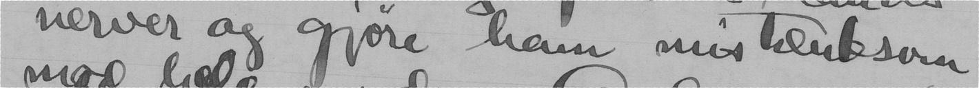nerver og gjøre ham mistænksom
